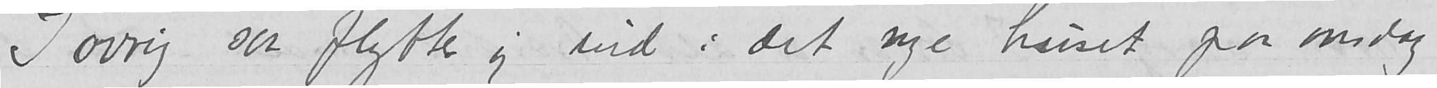I øvrig saa flytter jeg ind i det nye huset paa onsdag
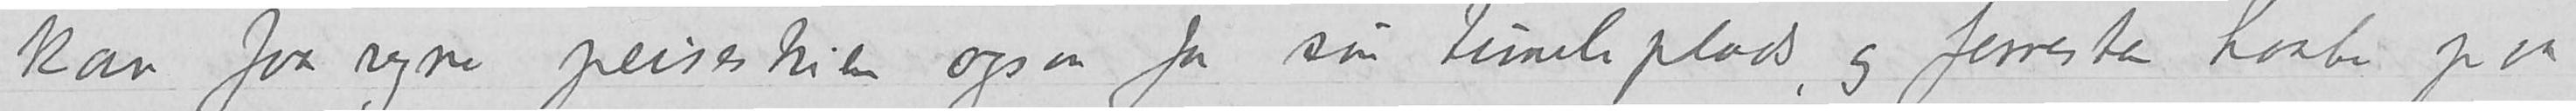kan faa regne peisestuen ogsaa for sin tumleplads, og forresten haaber paa
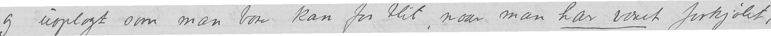og uoplagt som man bare kan faa blit, naar man har været forkjølet,
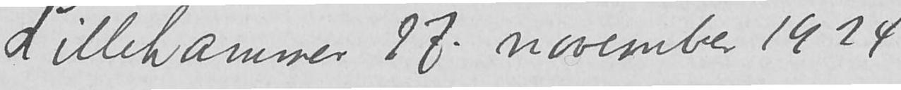Lillehammer 27. november 1924.
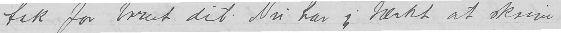tak for brevet dit. Nu har jeg tænkt at skrive
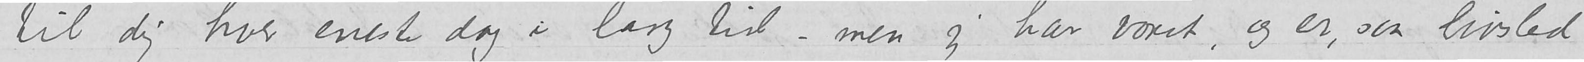til dig hver eneste dag i lang tid – men jeg har været, og er, saa livsled
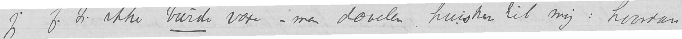jeg f.t. ikke burde være – men dævelen hvisker til mig: hvordan
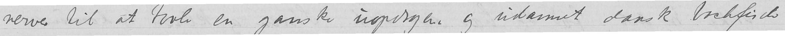nerver til at taale en ganske uopdragen og udannet dansk backfisch
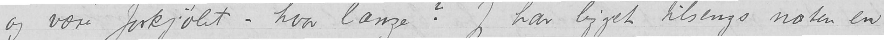og være forkjølet – hvor længe? Jeg har ligget til sengs næsten en
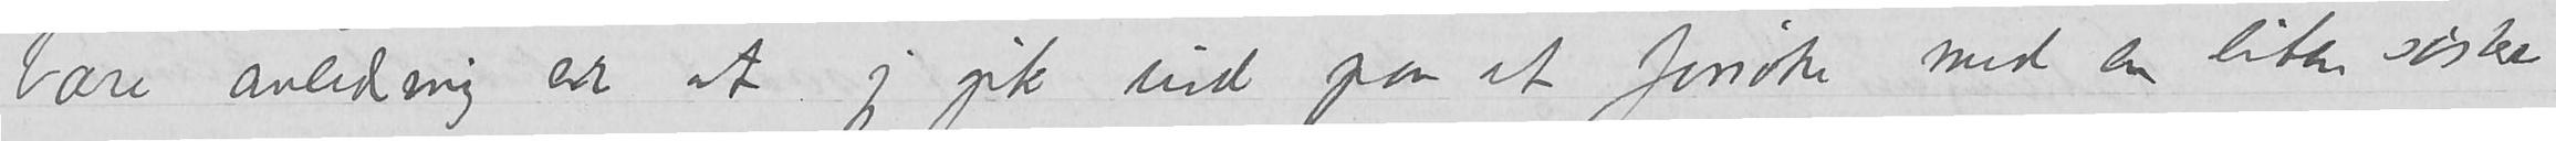bare anledning er at jeg gik ind paa et forsøk med en liten søster
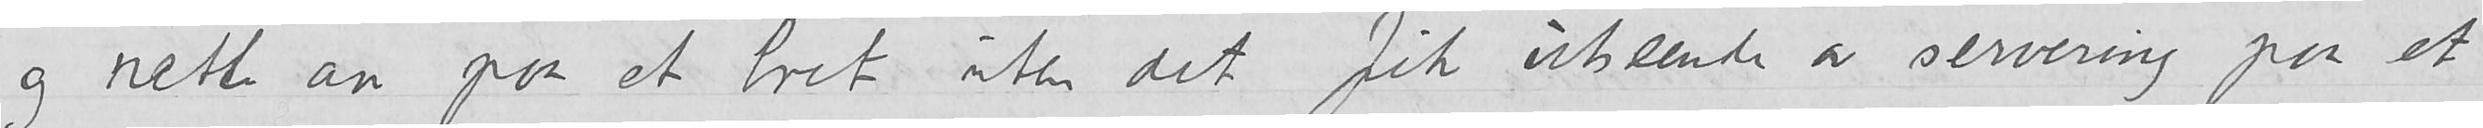og rette an paa et bret uten det fik utseende av servering paa et
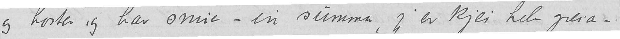og hoster og har snue – in summa, jeg er kjei hele greia –.
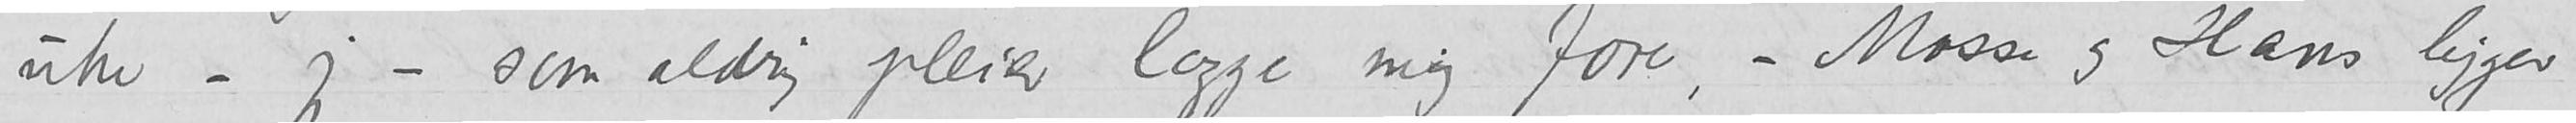uke – jeg – som aldrig pleier legge mig fore, – Mosse og Hans ligger
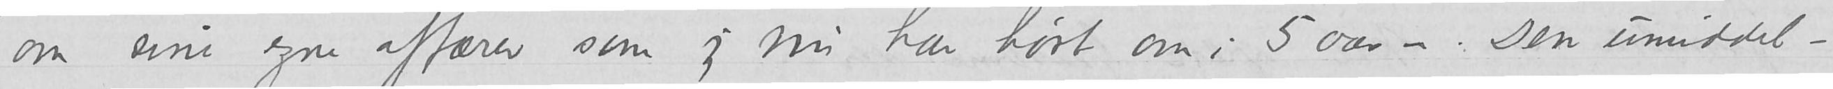om sine egne affærer som jeg nu har hørt om i 5 aar –. Den umiddel-
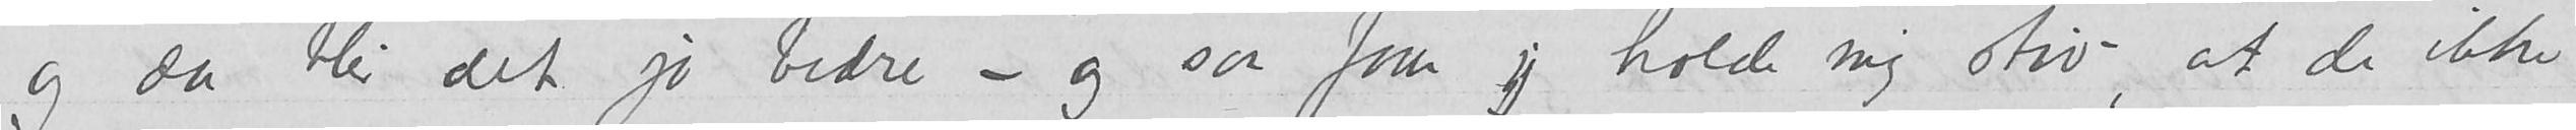og da blir det jo bedre – og saa faar jeg holde mig stiv –, at de ikke
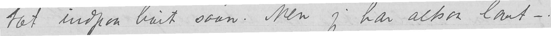tæt indpaa livet saan. Men jeg har altsaa lovet –.
